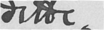dette
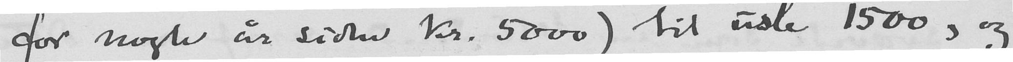for nogle år siden kr. 5000) til usle 1500, og
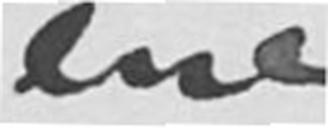ene
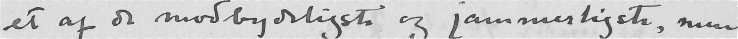et af de modbydeligste og jammerligste, men
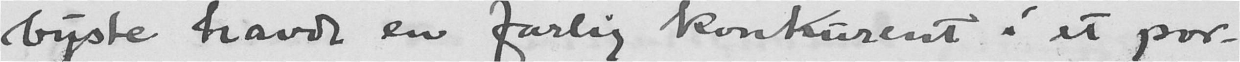byste havde en farlig konkurent i et por-
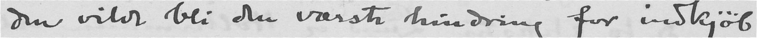den vilde bli den værste hindring for indkjøb
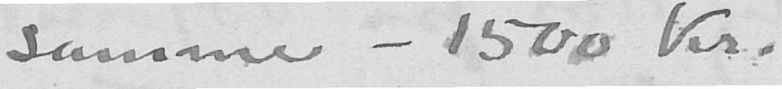samme - 1500Kr.
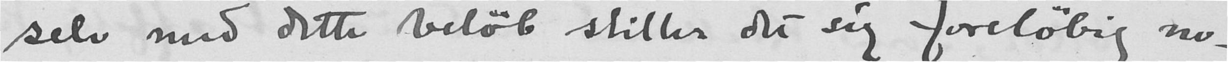selv med dette beløb stiller det sig foreløbig no
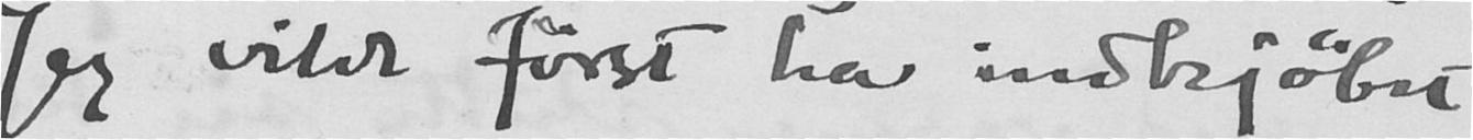Jeg vilde først ha indkjøbet
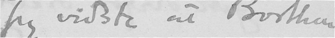Jeg vidste at Borthen
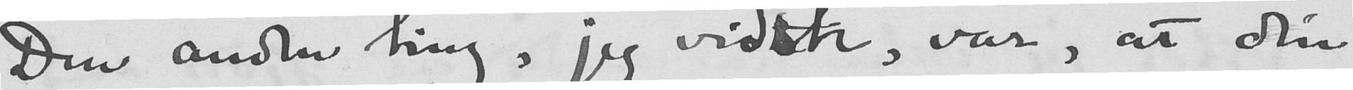Den anden ting, jeg vidste, var, at din
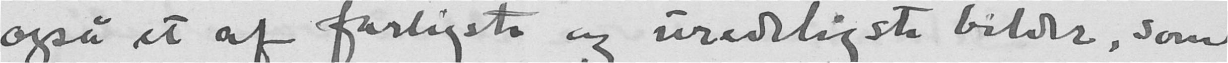også et af farligste og uredeligste bilder, som
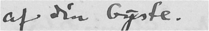af din byste.
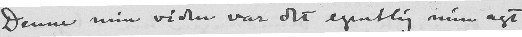Denne min viden var det egentlig min agt
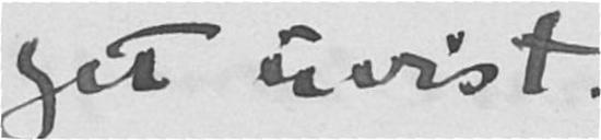get uvist.
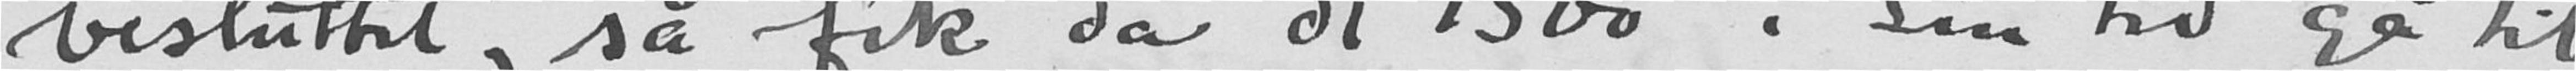besluttet, så fik da de 1500 i sin tid gå til
